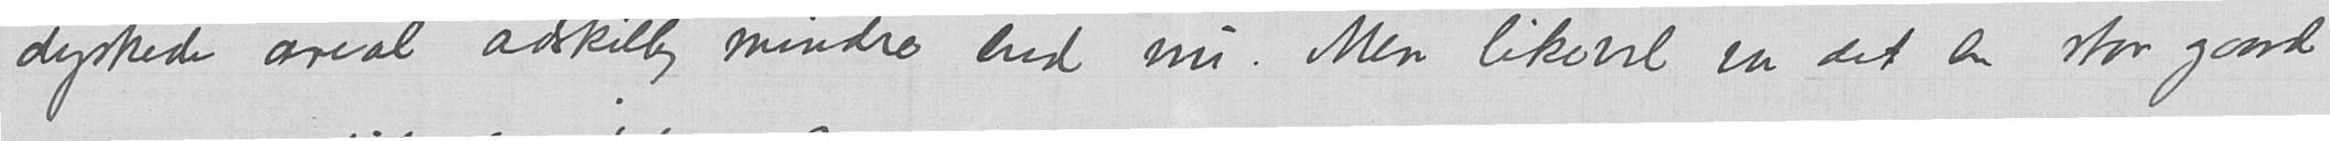dyrkede areal adskillig mindre end nu. Men likevel var det en stor gaard
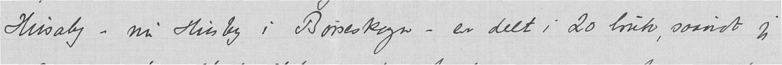Husaby – nu Husby i Børseskogn - er delt i 20 bruk, saavidt jeg
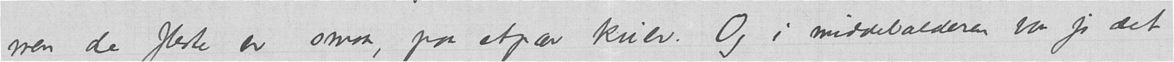men de fleste er smaa, paa etpar kuer. Og i middelalderen var jo det
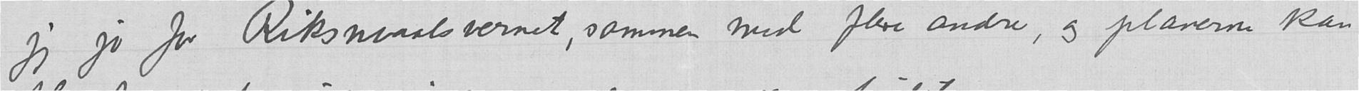jeg jo for Riksmaalsvernet, sammen med flere andre, og planerne kan
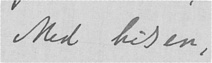Med hilsen,
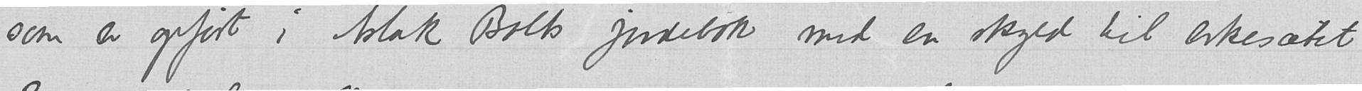som er opført i Aslak Bolts jordebok med en skyld til erkesætet
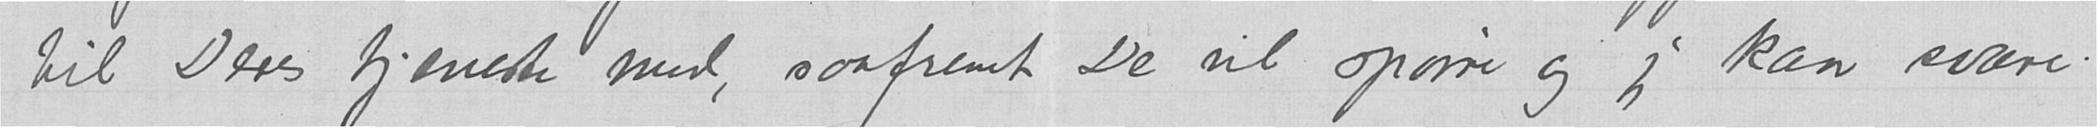til Deres tjeneste med, saafremt De vil spørre og jeg kan svare.
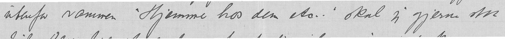utenfor rammen "Hjemme hos dem etc." skal jeg gjerne staa
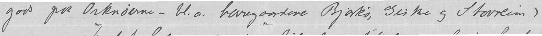gods paa Orknøerne – bl.a. herregaardene Bjarkø, Giske og Stavreim)
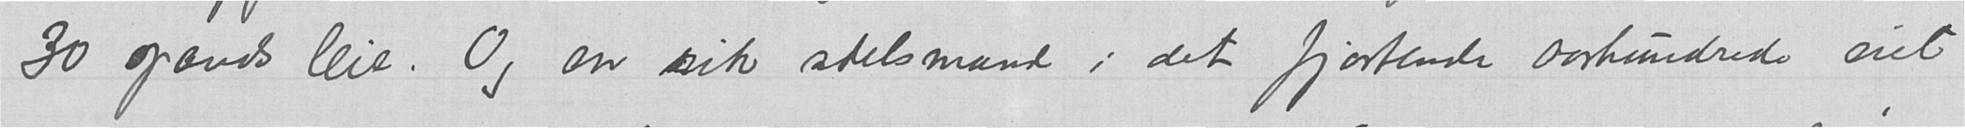30 spands leie. Og en slik adelsmand i det fjortende aarhundrede eiet
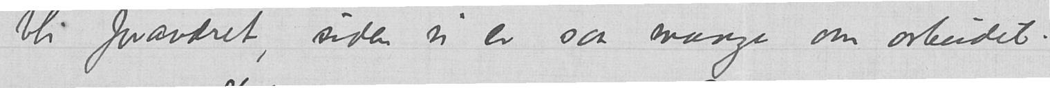bli forandret, siden vi er saa mange om arbeidet.
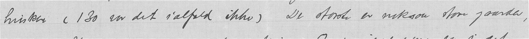husker (130 var det ialfald ikke) De største er noksaa store gaarder,
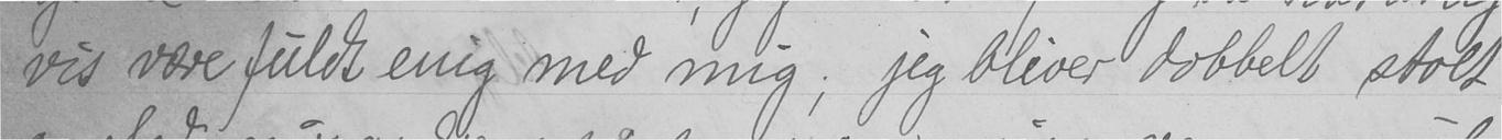vis være fuldt enig med mig; jeg bliver dobbelt stolt
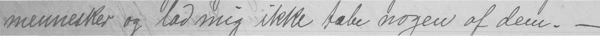mennesker og lad mig ikke tabe nogen af dem. -
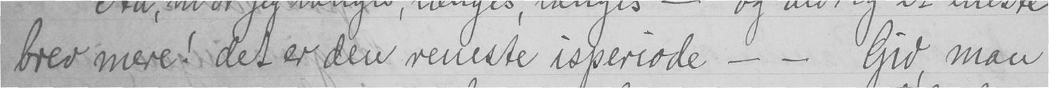brev mere! det er den reneste isperiode - - Gid, man
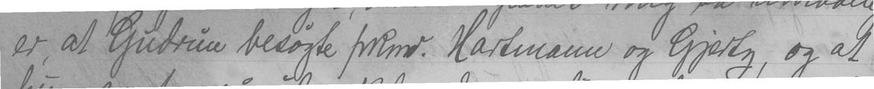er, at Gudrun besøgte frknr. Hartmann og Gjertz, og at
